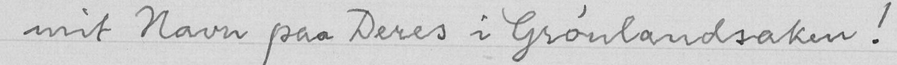mit Navn paa Deres i Grønlandsaken!
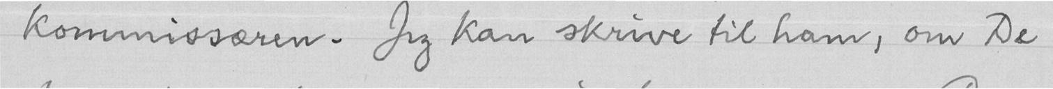kommissæren. Jeg kan skrive til ham, om De
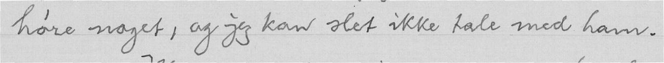høre noget, og jeg kan slet ikke tale med ham.
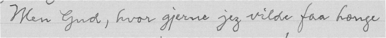Men Gud, hvor gjerne jeg vilde faa hænge
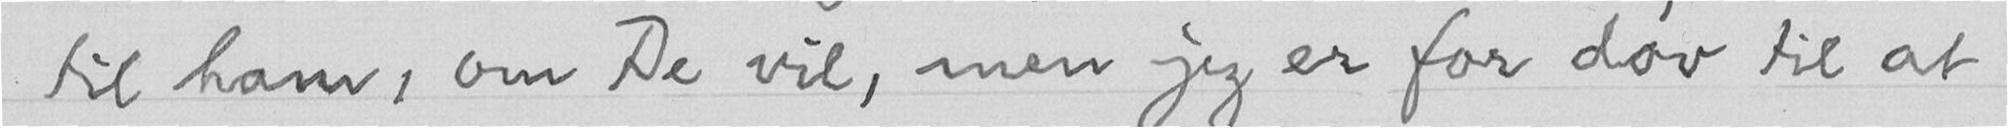til ham, om De vil, men jeg er for døv til at
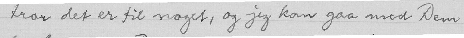tror det er til noget, og jeg kan gaa med Dem
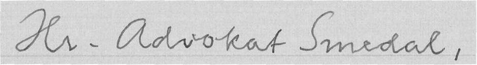Hr. Advokat Smedal,
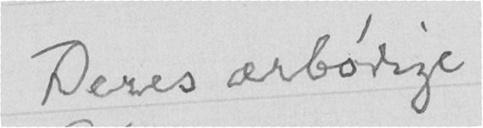Deres ærbødige
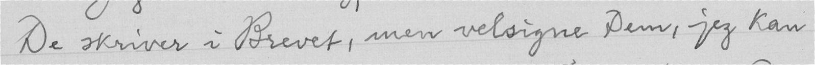De skriver i Brevet, men velsigne Dem, jeg kan
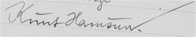Knut Hamsun.
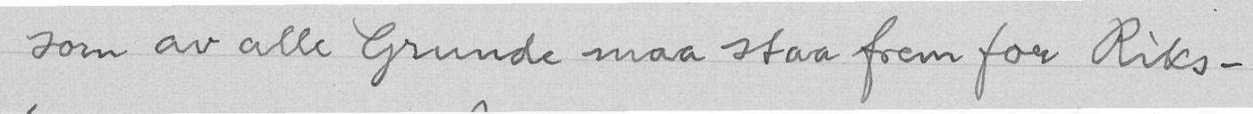som av alle Grunde maa staa frem for Riks-
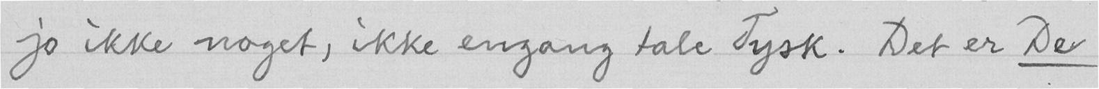jo ikke noget, ikke engang tale Tysk. Det er De
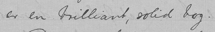er en brilliant, solid bog.
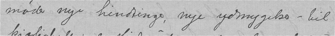møder nye hindringer, nye ydmygelser - til
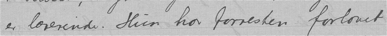er lærerinde. Hun har forresten forlovet
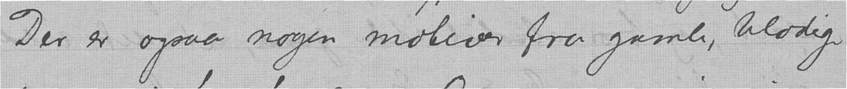Der er ogsaa nogen motiver fra gamle, blodige
